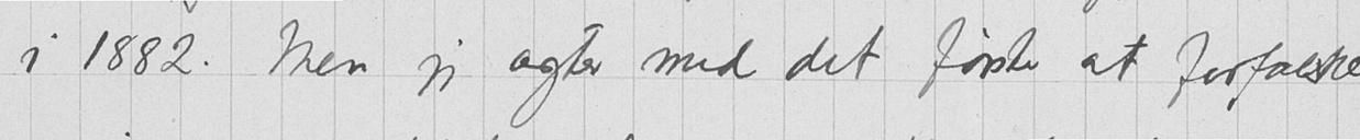i 1882. Men jeg agter med det første at forfalske
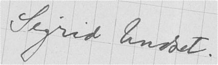Sigrid Undset.
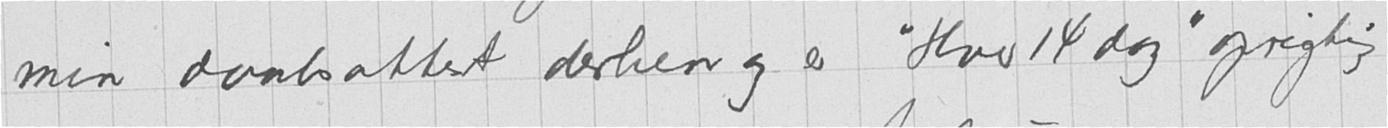min daabsattest derhen og er "Hver14dag" oprigtig
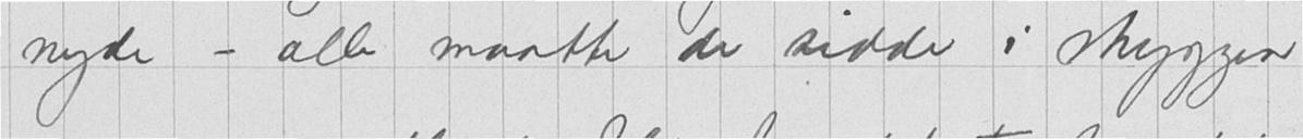nyde – alle maatte de sidde i skyggen
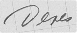Deres
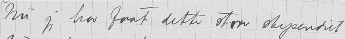Nu jeg har faat dette store stipendiet
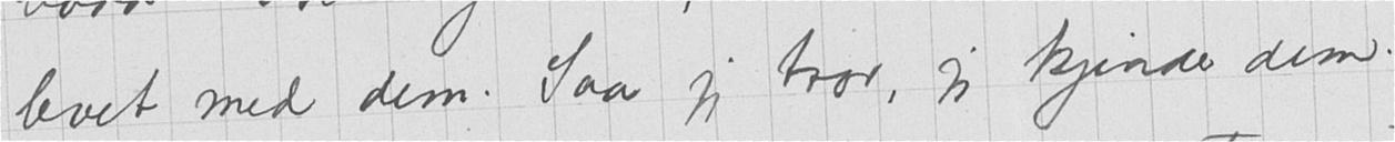levet med dem. Saa jeg tror, jeg kjender dem.
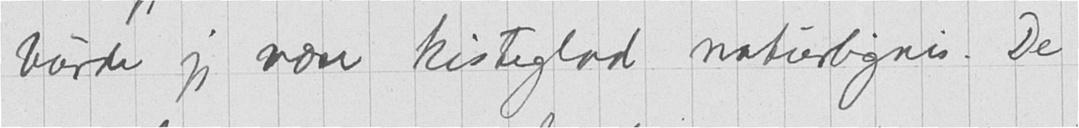burde jeg være kisteglad naturligvis. De
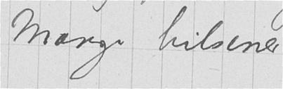Mange hilsener
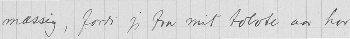mæssig, fordi jeg fra mit tolvte aar har
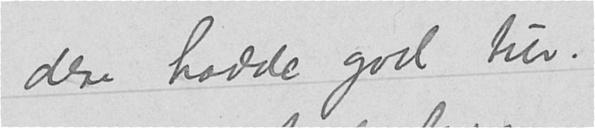dere hadde god tur.
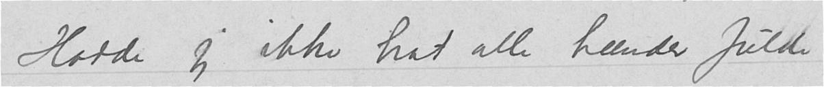Hadde jeg ikke hat alle hænder fulde
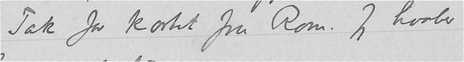Tak for kortet fra Rom. Jeg haaber
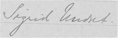Sigrid Undset.
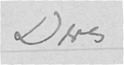Deres
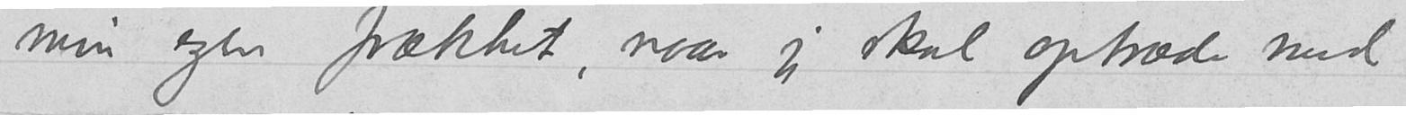min egen frækhet, naar jeg skal optræde med
